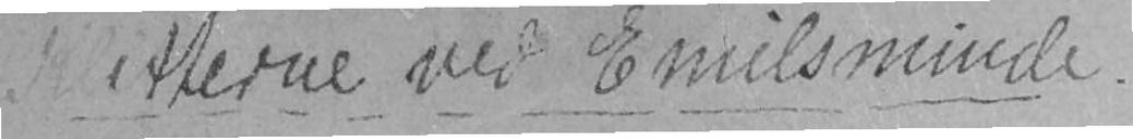Klitterne ved Emilsminde.
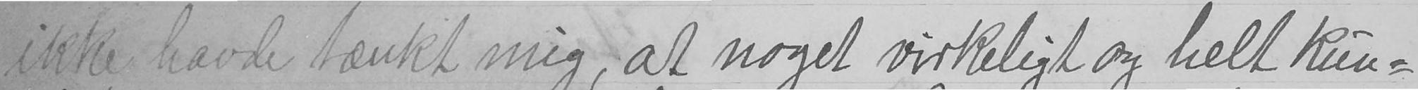ikke havde tænkt mig, at noget virkeligt og helt kun-
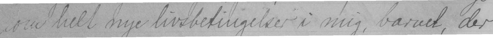som helt nye livsbetingelser i mig, barnet, der
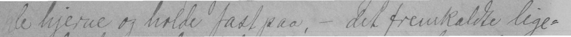e hjerne og holde fast paa, - det fremkaldte lige-
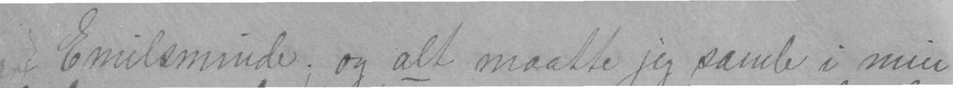Emilsminde og alt maatte jeg samle i min
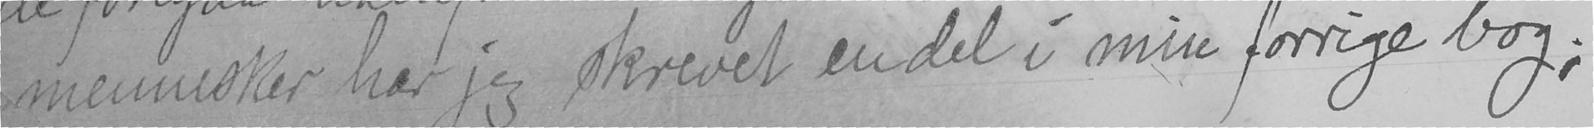mennesker har jeg skrevet endel i min forrige bog;
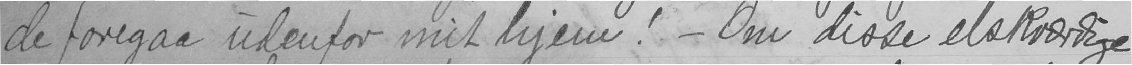de foregaa udenfor mit hjem! - Om disse elskværdige
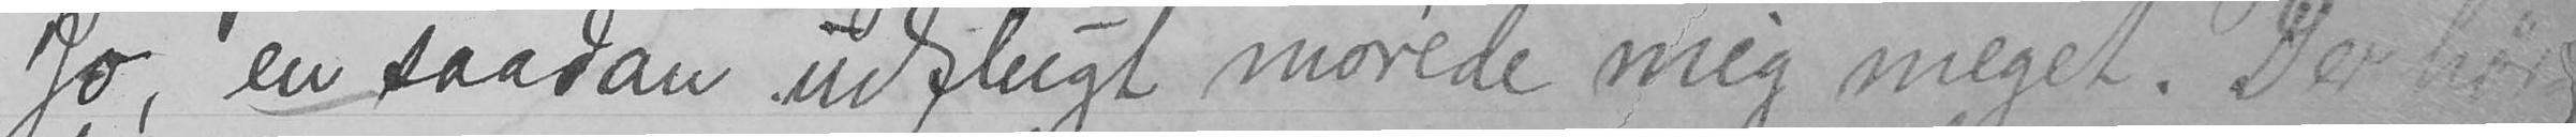Jo, en saadan udflugt morede mig meget. Der hør-
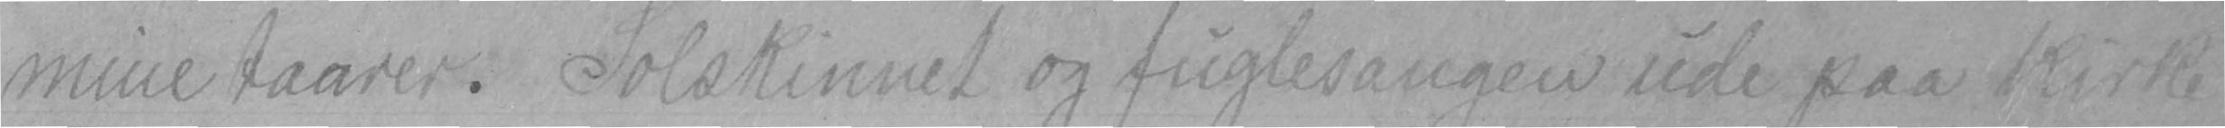mine taarer. Solskinnet og fuglesangen ude paa kirke-
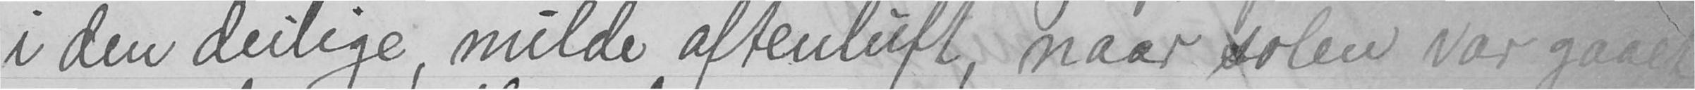i den deilige, milde aftenluft, naar solen var gaaet
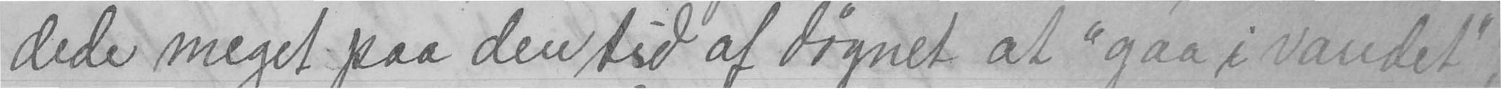dede meget paa den tid af døgnet at "gaa i vandet",
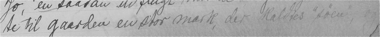te til gaarden en stor mark, der kaldtes "søen", og
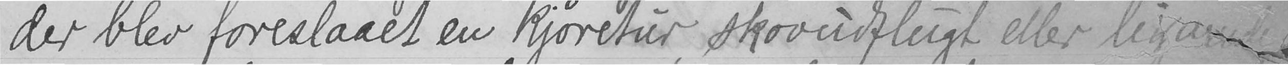der blev foreslaaet en kjøretur, skovudflugt eller li
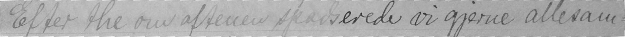Efter the om aftenen spadserede vi gjerne allesam-
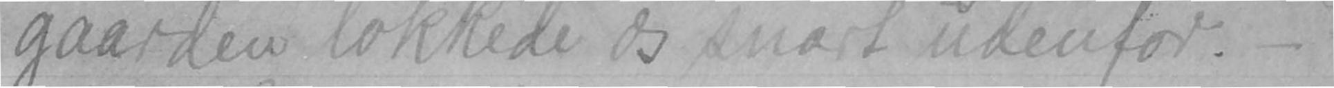gaarden lokkede os snart udenfor. -
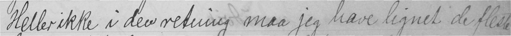Heller ikke i den retning maa jeg have lignet de fleste
